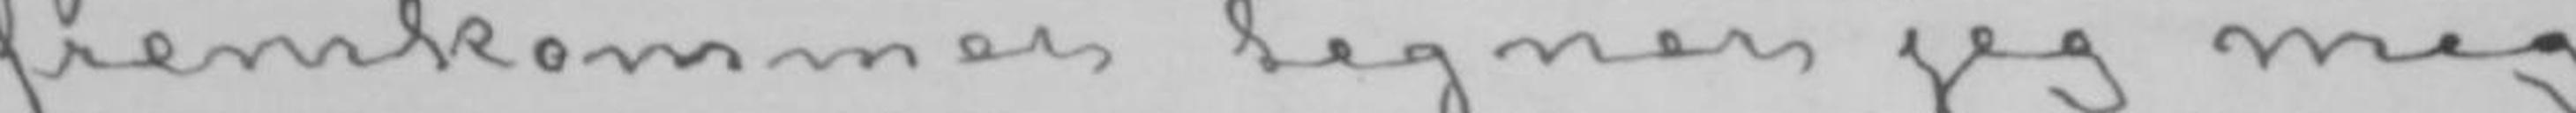fremkommer tegner jeg mig
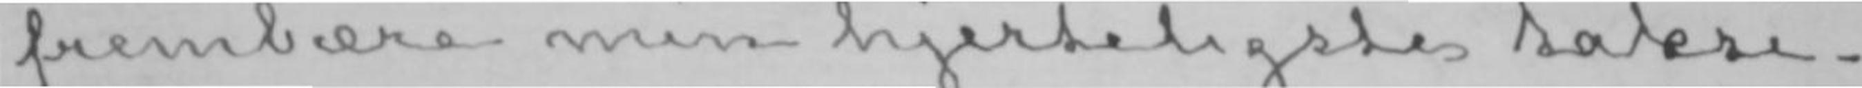frembære min hjerteligste taksi-
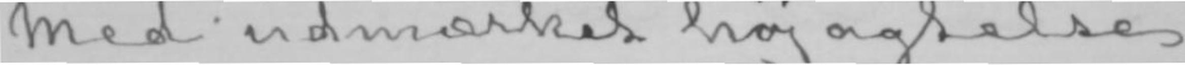Med udmærket højagtelse
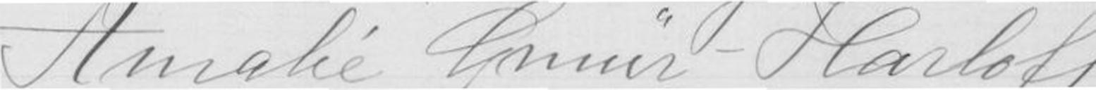Amalie Gmür-Harloff
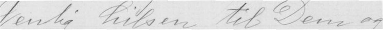Venlig hilsen til Dem og
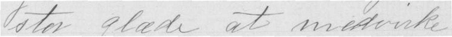stor glæde at medvirke
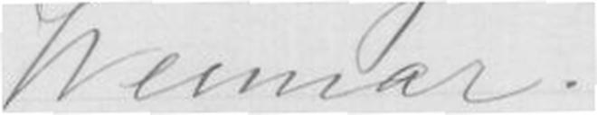Weimar
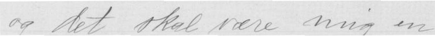og det skal være mig en
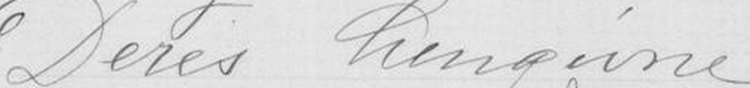Deres hengivne
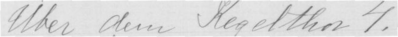Über dem Kegelthon 4.
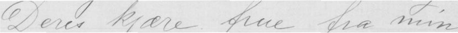Deres kjære frue fra min
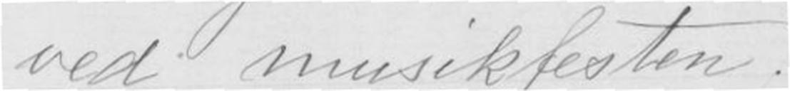ved musikfesten.
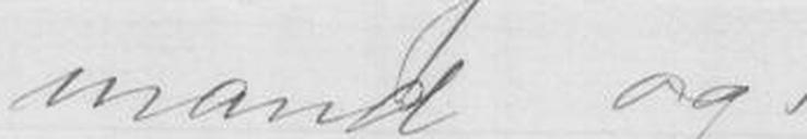mand og
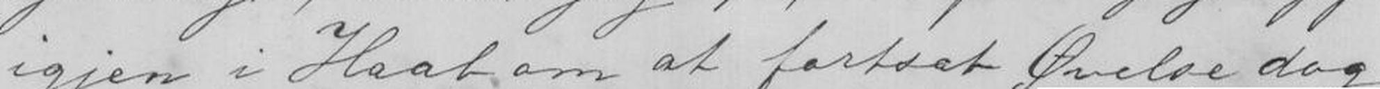igjen i Haab om at fortsat Øvelse dog
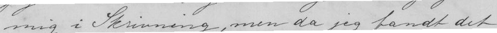mig i Skrivning, men da jeg fandt det
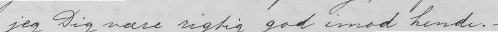jeg Dig være rigtig god imod hende.-
